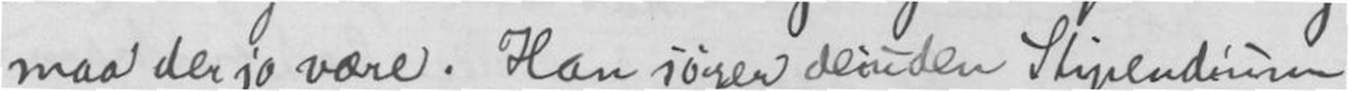maa der jo være. Han søger desuden Stipendium
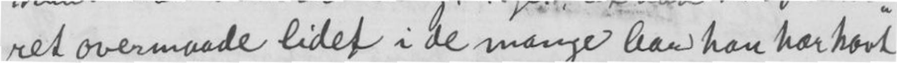ret overmaade lidet i de mange Aar han har havt
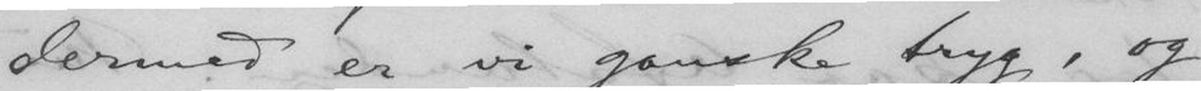dermed er vi ganske tryg, og
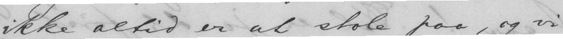ikke altid er at stole paa, og vi
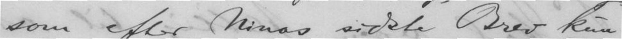som efter Ninas sidste Brev kun-
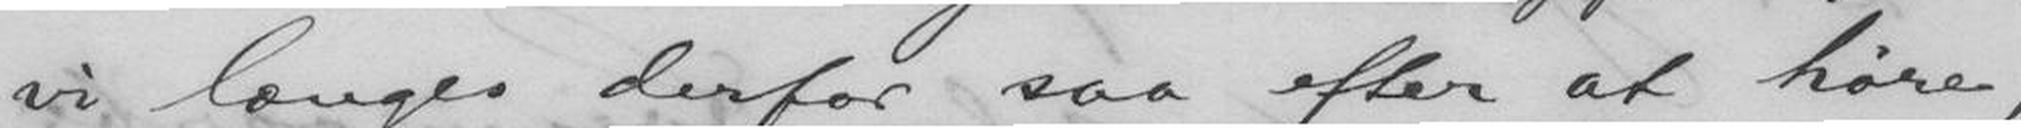vi længes derfor saa efter at høre,
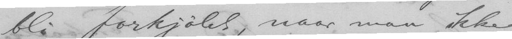bli forkjølet, naar man ikke
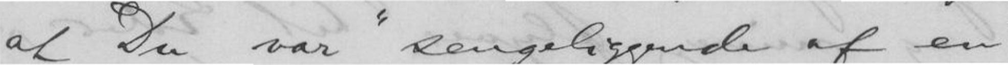at Du var "sengeliggende af en
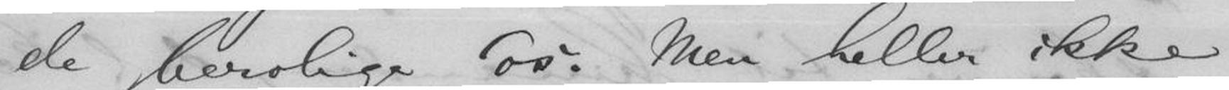de berolige os. Men heller ikke
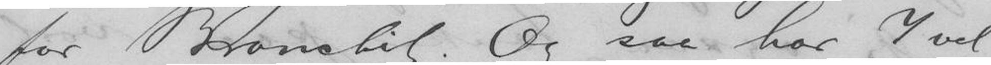for Bronchit. Og saa har I vel
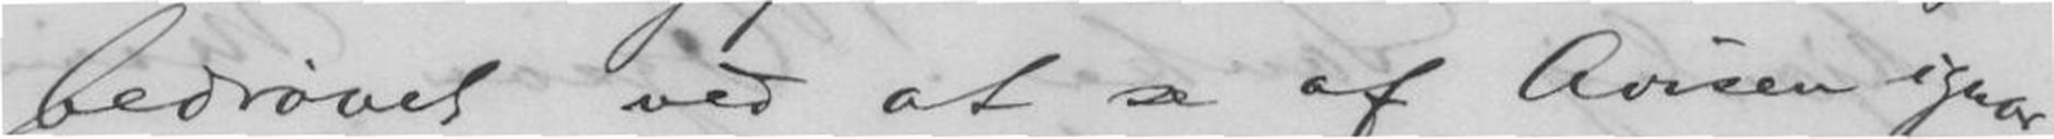bedrøvet ved at se af Avisen igaar,
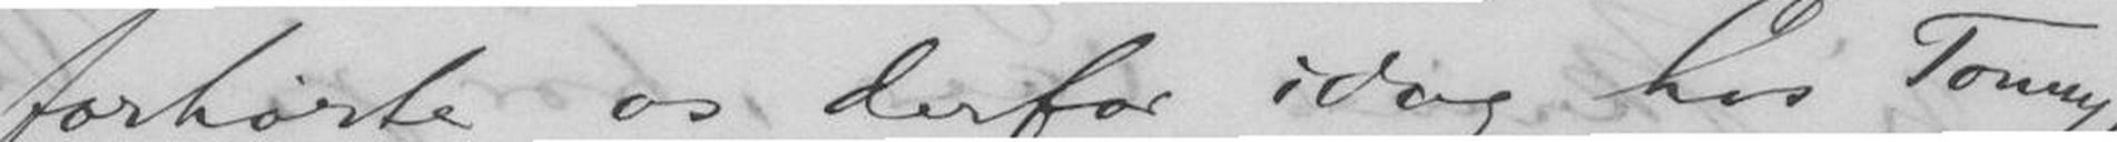forhørte os derfor idag hos Tonny,
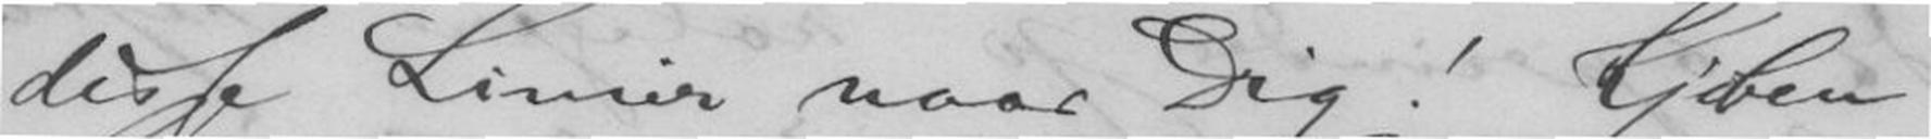disse Linier naar Dig! Kjøben-
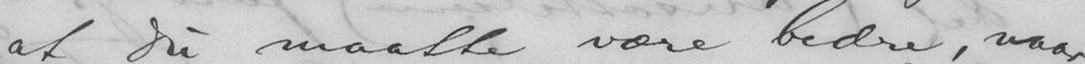at Du maatte være bedre, naar
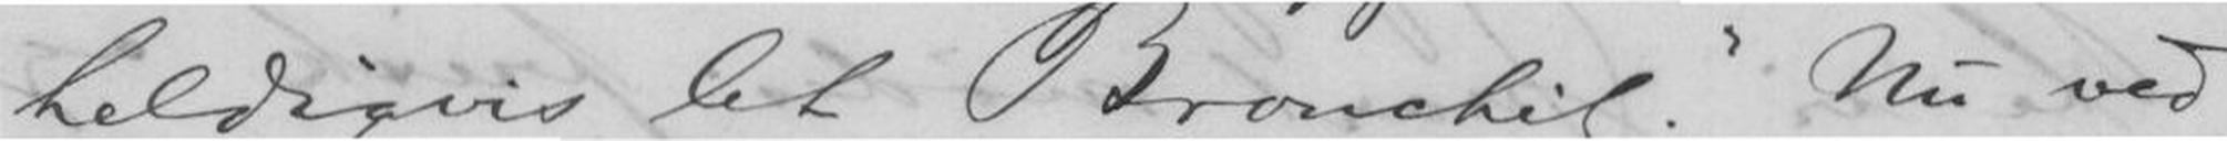heldigvis let Bronchit". Nu ved
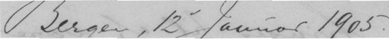Bergen, 12 Januar 1905
